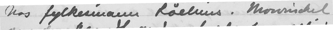hos fylkesmann Joachim. Mowinckel
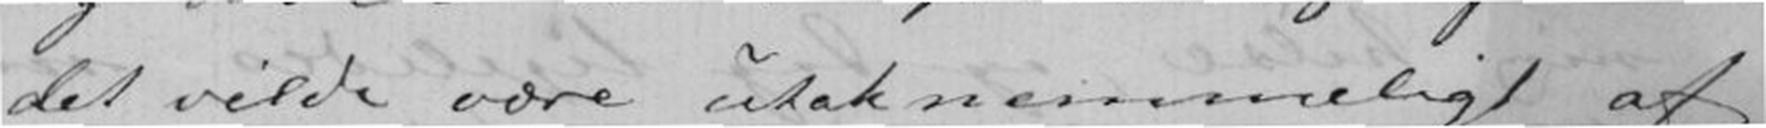det vilde være utaknemmeligt af
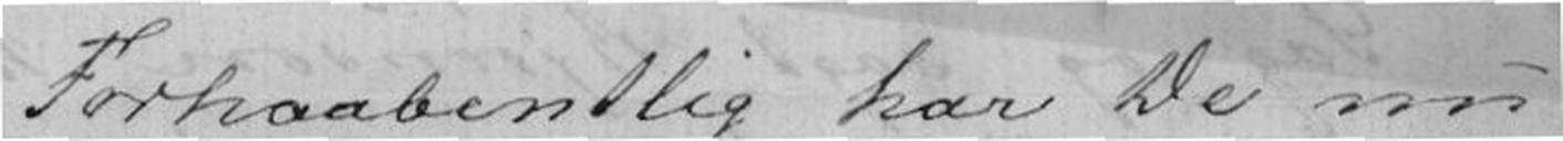Forhaabentlig har De nu
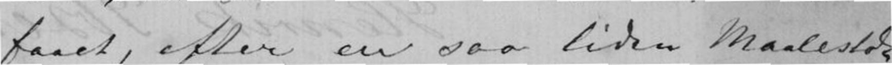faaet, efter en saa liden Maalestok
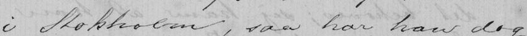i Stokholm, saa har han dog
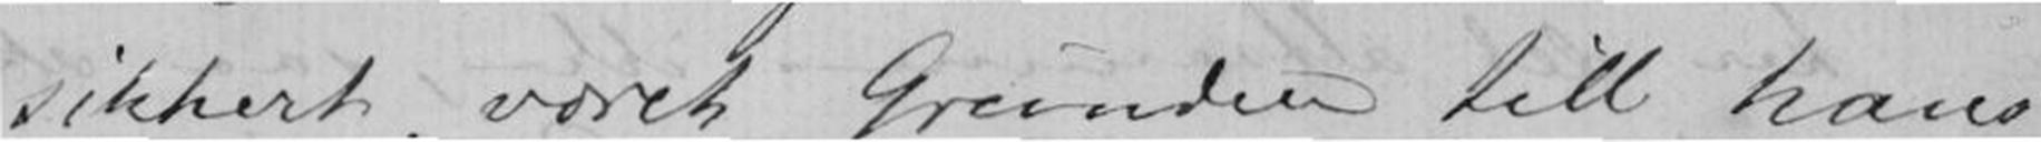sikkert været Grunden till hans
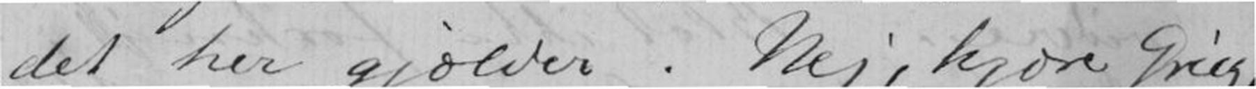det her gjælder. Nej, kjære Grieg,
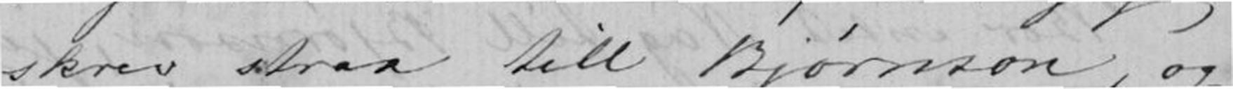skrev strax till Bjørnson, og
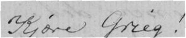Kjære Grieg!
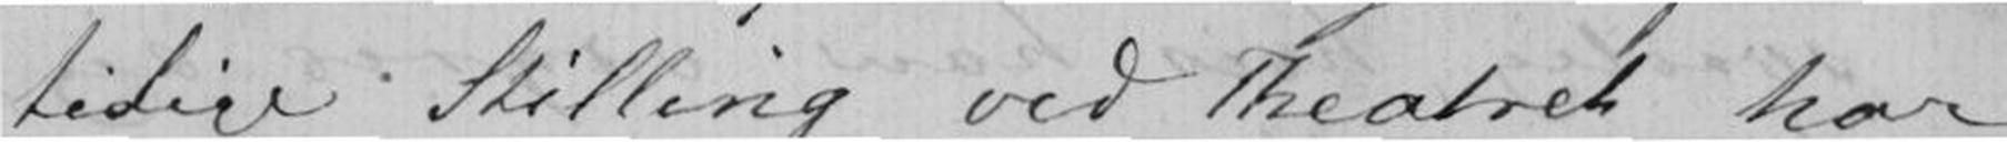tidige Stilling ved Theatret har
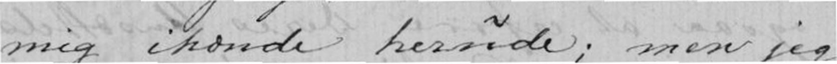mig ihænde herude; men jeg
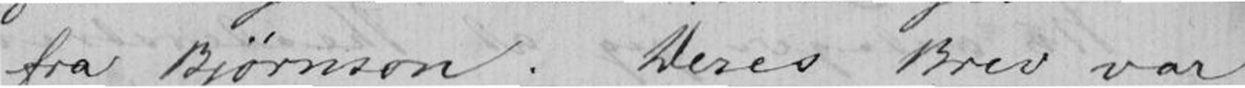fra Bjørnson. Deres Brev var
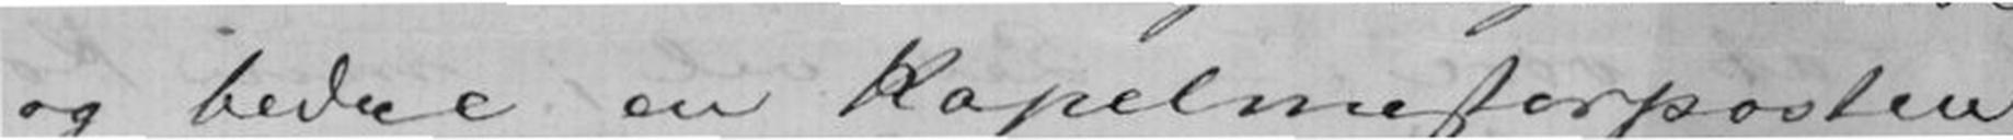og bedre en Kapelmesterposten,
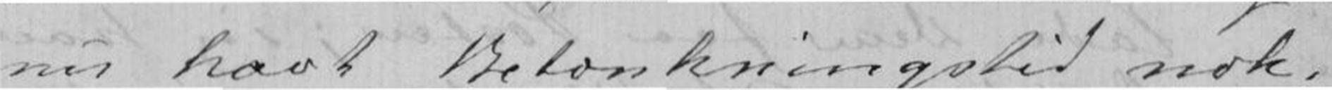nu havt Betænkningstid nok.
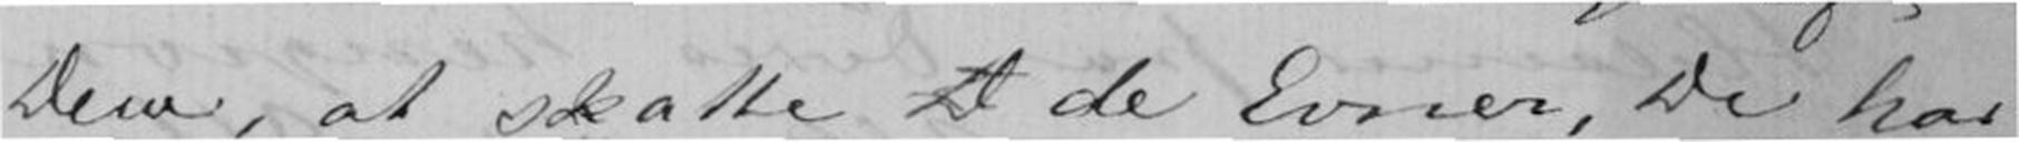Dem, at skatte D de Evner, De har
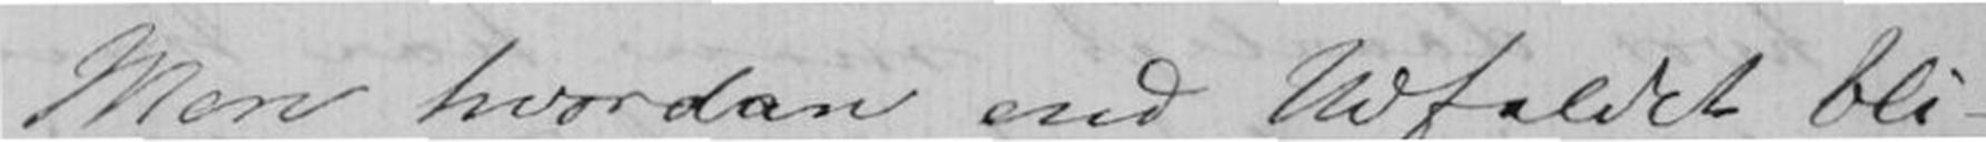Men hvordan end Udfaldet bli-
